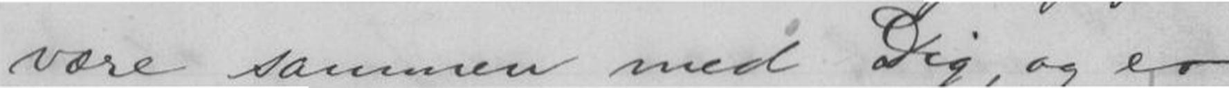være sammen med Dig, og er
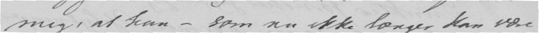mig, at han - som nu ikke længer kan være
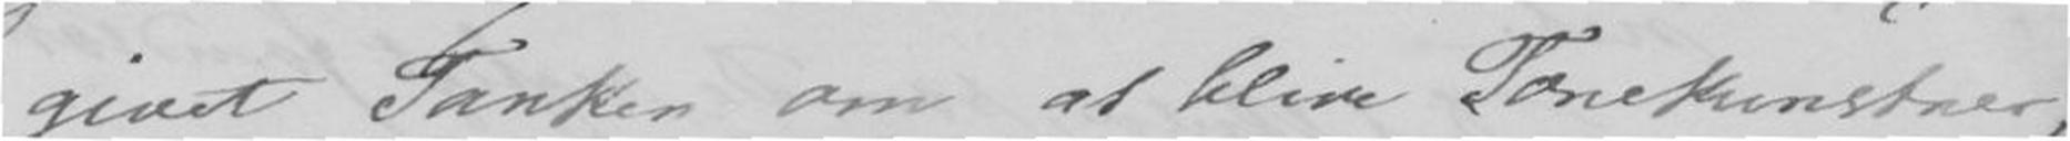givet Tanken om at blive Tonekunstner,
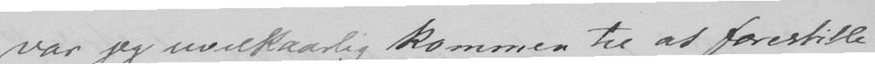var jeg vilkaarlig kommen til at forestille
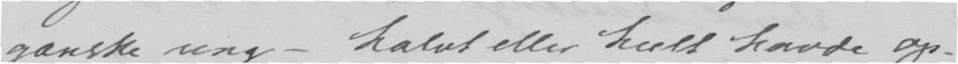ganske ung - halvt eller helt havde op-
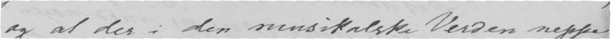og at der i den musikalske Verden neppe
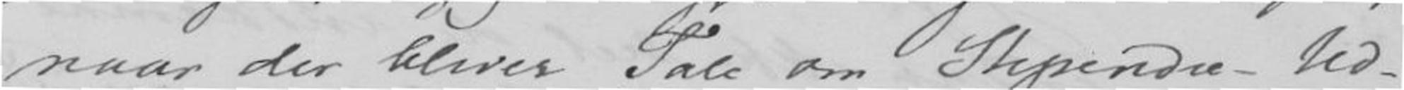naar der bliver Tale om Stipender - Ud-
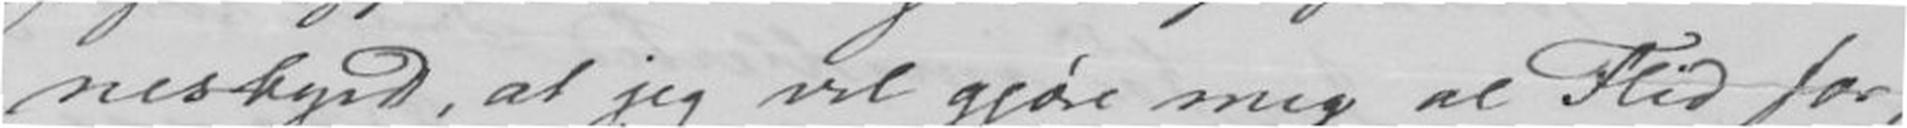nesbyrd, at jeg vil gjøre mig al Flid for,
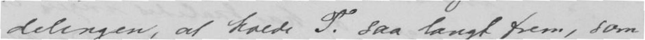delinger, at holde T. saa langt frem, som
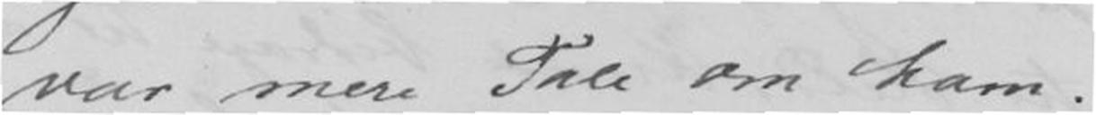var mere Tale om ham.
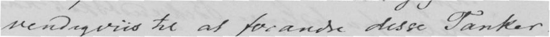vendigvis til at forandre disse Tanker.
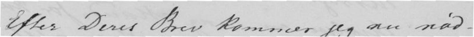Efter Deres Brev kommer jeg om nød-
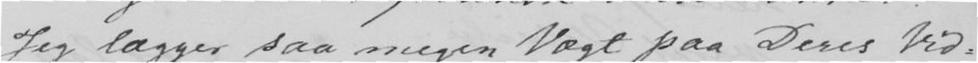Jeg lægger saa megen Vægt paa Deres Vid-
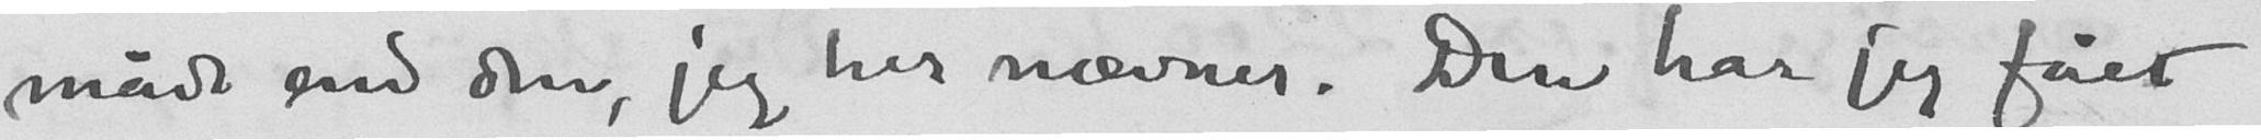måde end den, jeg her nævner. Den har jeg fået
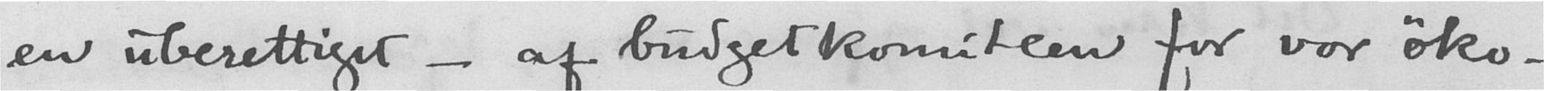en uberettiget - af budgetkommiteen for vor øko-
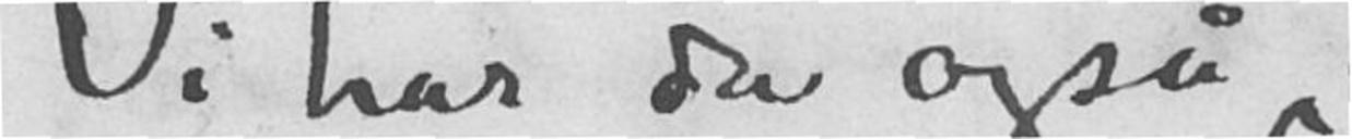Vi har da også
